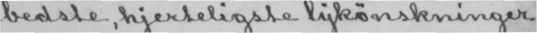bedste, hjerteligste lykønskninger
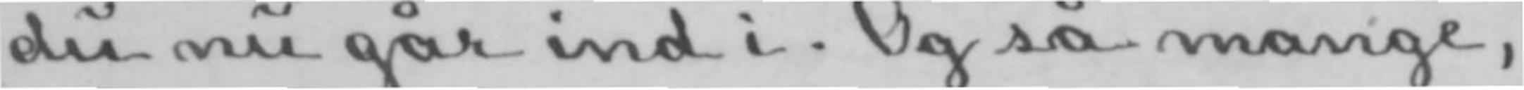du nu går ind i. Og så mange,
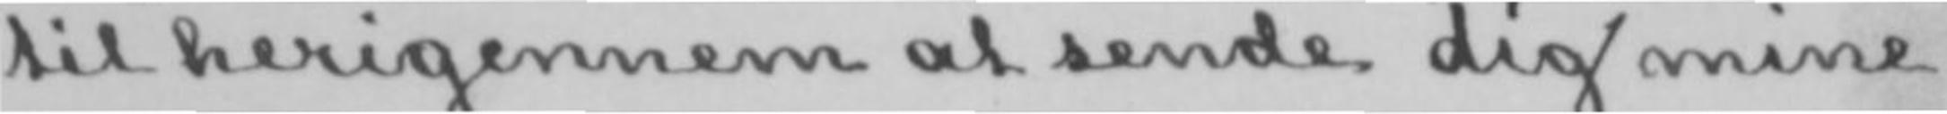til herigennem at sende dig mine
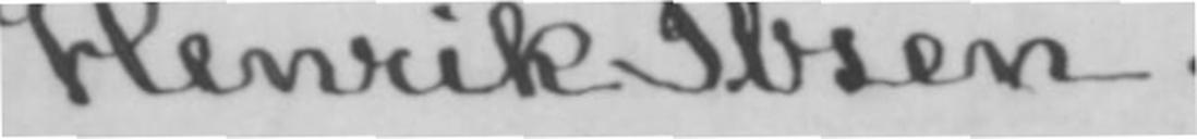Henrik Ibsen.
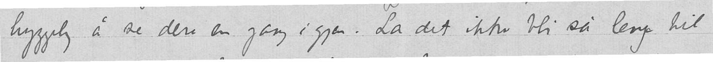hyggelig å se dere en gang igjen. La det ikke bli så lenge til
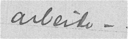arbeide – .
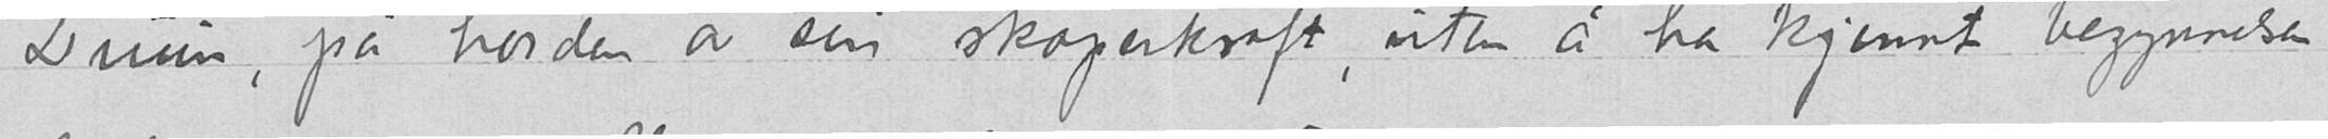Duun, på høiden av sin skaperkraft, uten å ha kjennt begynnelsen
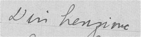Din hengivne
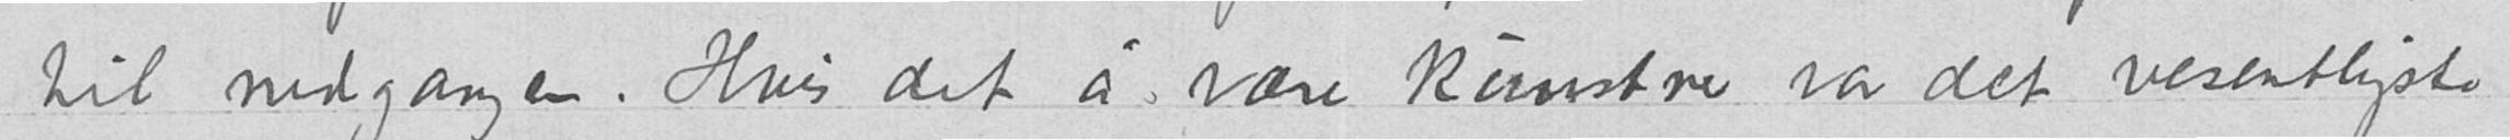til nedgangen. Hvis det å være kunstner var det vesentligste
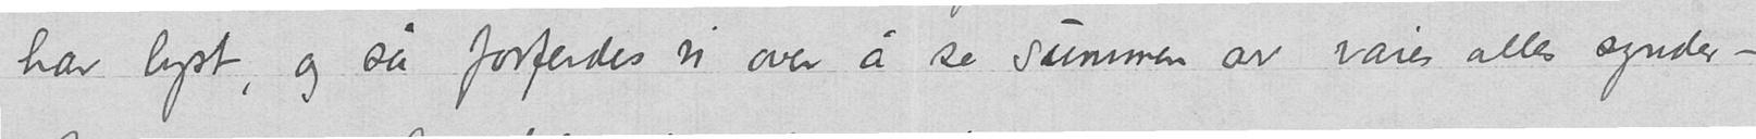har lyst, og så forferdes vi over å se summen av våre alles synder – .
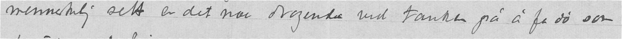menneskelig sett er det noe dragende ved tanken på å få dø som
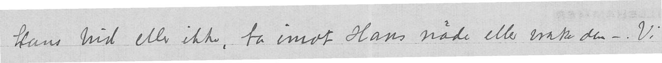Hans bud eller ikke, ta imot Hans nåde eller vrake den –. Vi
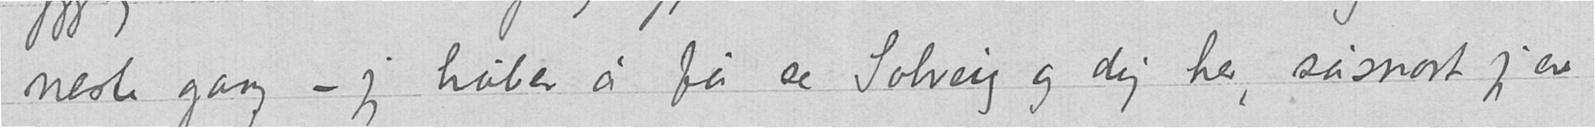neste gang – jeg håber å få se Solveig og dig her, såsnart jeg er
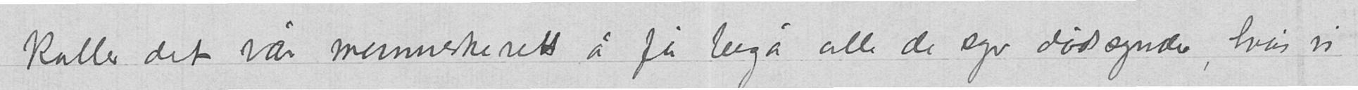kaller det vår menneskerett å få begå alle de syv dødssynder, hvis vi
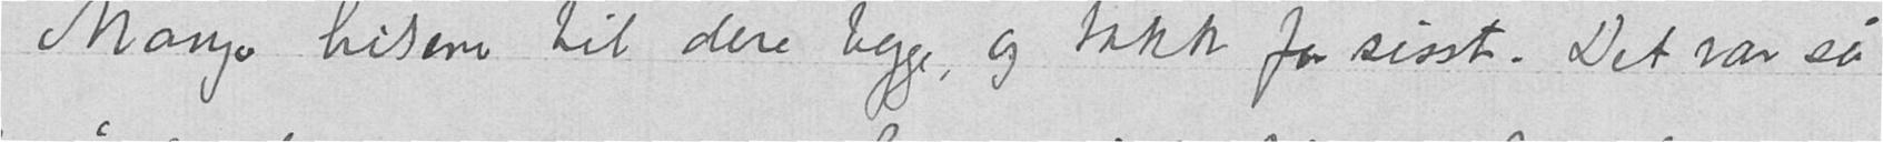Mange hilsener til dere begge, og takk for sisst. Det var så
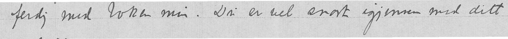ferdig med boken min. Du er vel snart igjennem med ditt
